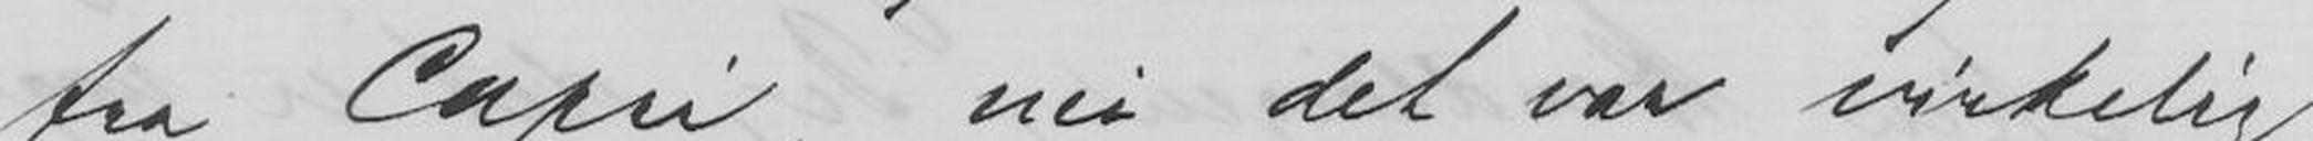fra Capri, nei det var virkelig
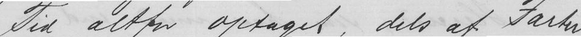Tid altfor optaget, dels af Farter
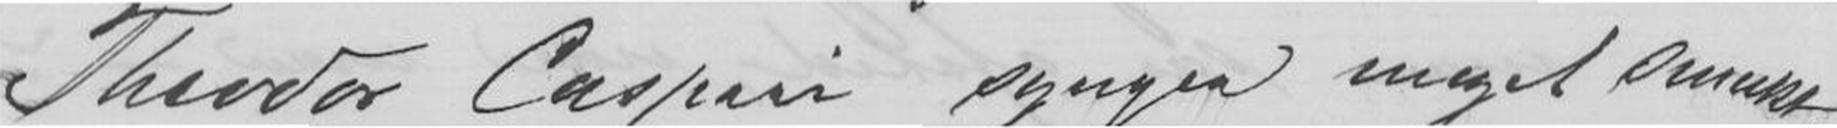Theodor Caspari synger meget smukt,
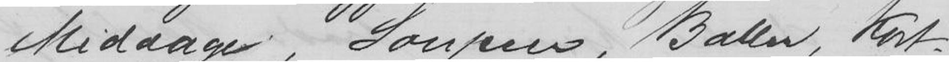Middage, Soupeer, Baller, Kort-
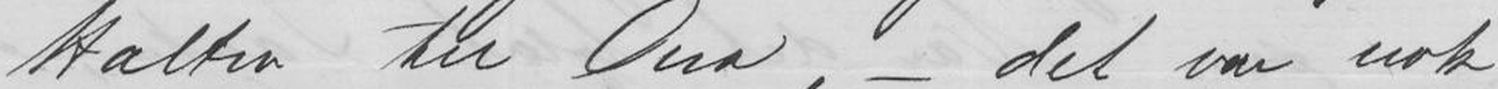Halten til Ona, - det var nok
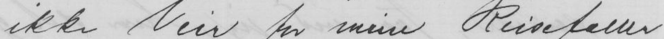ikke Veir for mine Reisefæller
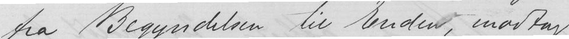fra Begyndelsen til Enden, modtog
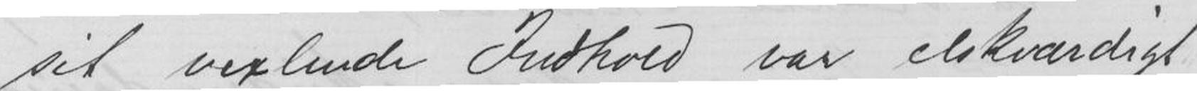sit vexlende Indhold var elskværdigt
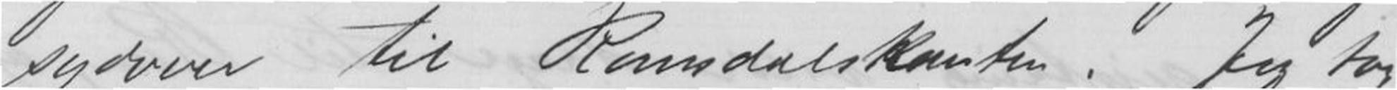sydover til Romsdalskanten. Jeg tog
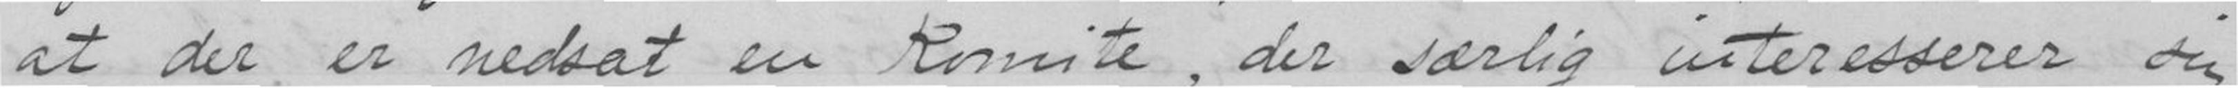at der er nedsat en Komite, der særlig interesserer sig
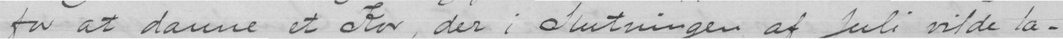for at danne et Kor, der i Slutningen af Juli vilde la-
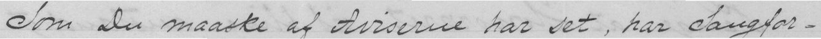Som Du maaske af Aviserne har set, har Sangfor-
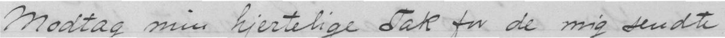Modtag min hjertelig Tak for de mig sendte
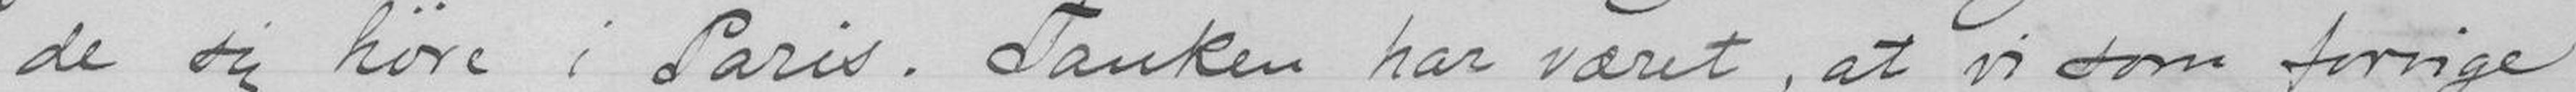de sig høre i Paris. Tanken har været, at vi som forrige
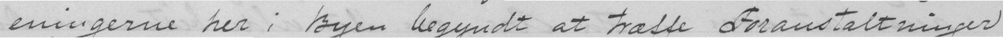eningerne her i Byen begyndt at træffe Foranstaltninger
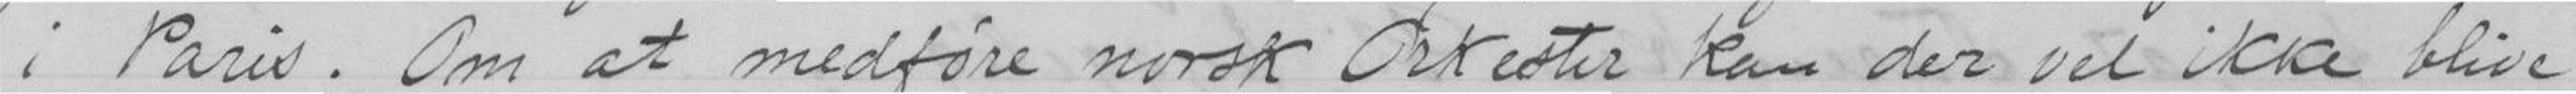i Paris. Om at medføre norsk Orkester Rom der vil ikke blive
